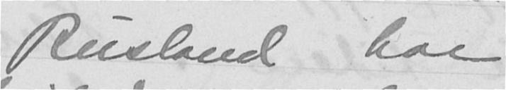Rusland har
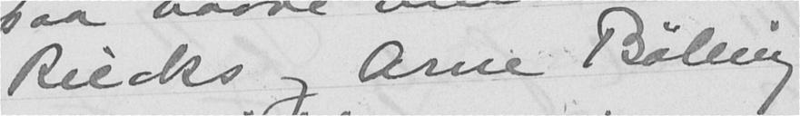Riecks og Arne Bølling
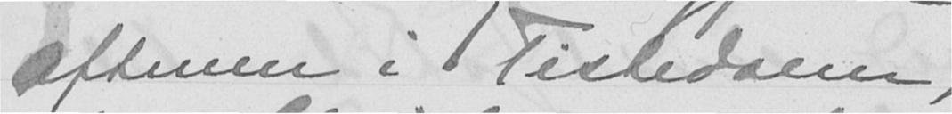aftenen i Tistedalen,
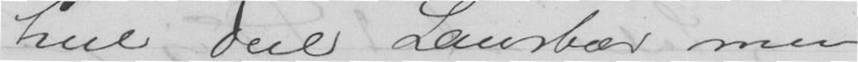heel Deel Laurbær men
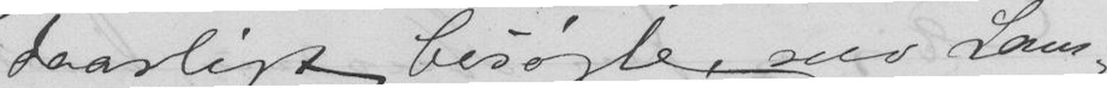daarligt besøgte, selv Lam-
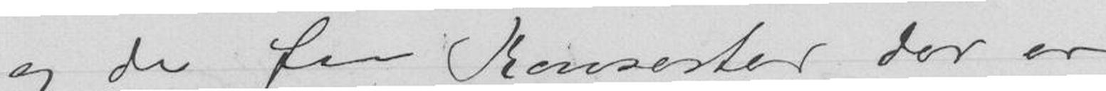og de faa Konserter der er
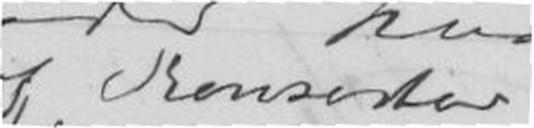af Konserter
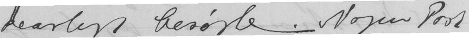Daarligt besøgte. Nogen Post
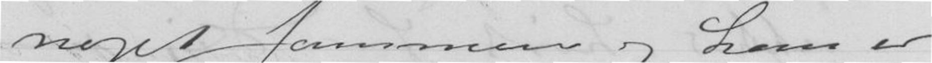noget sammen og ham er
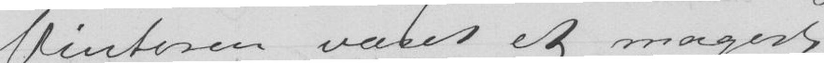Vinteren varet et magert
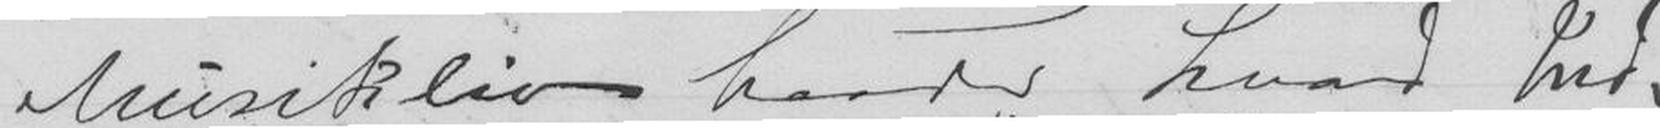Musikliv baade hvad Ind-
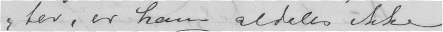ter, er ham aldeles ikke
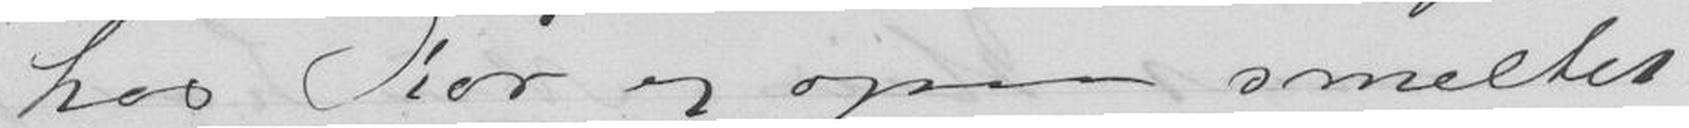hos Kor og ogsaa smeltet
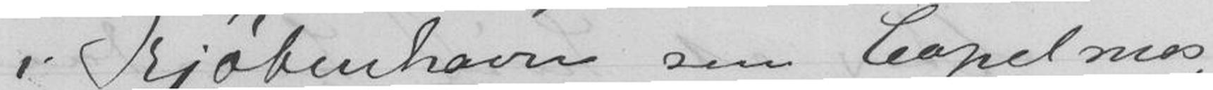i Kjøbenhavn som Capelmes-
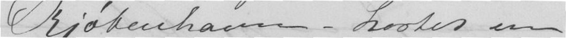Kjøbenhavn - høstet en
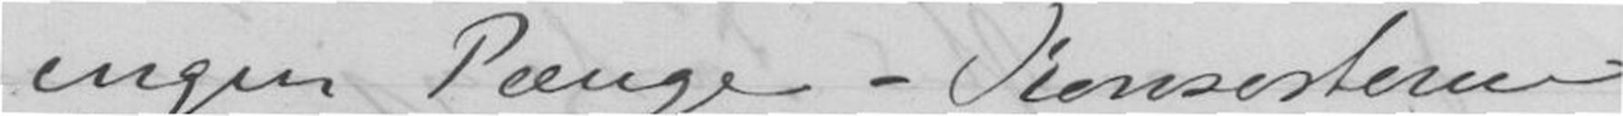ingen Pænge - Konserterne
